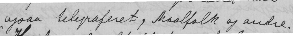ogsaa telegraferet, Maalfolk og andre.
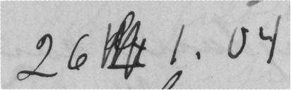26  1.04
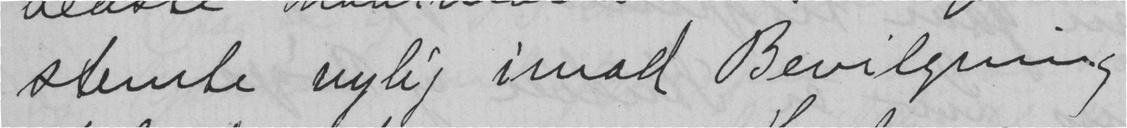stemte nylig imod Bevilgning
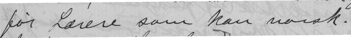for Lærere som kan norsk.
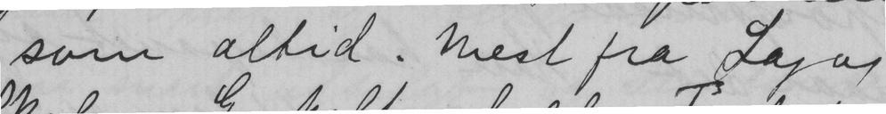som altid. Mest fra Lag og
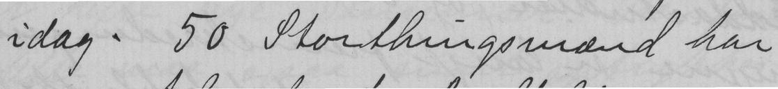idag. 50 Storthingsmænd har
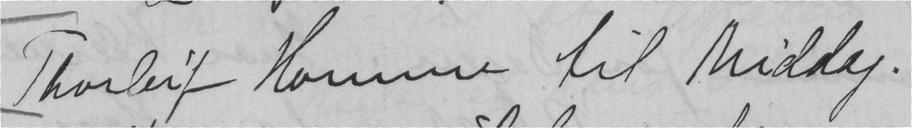Thorleif Homme til middag.
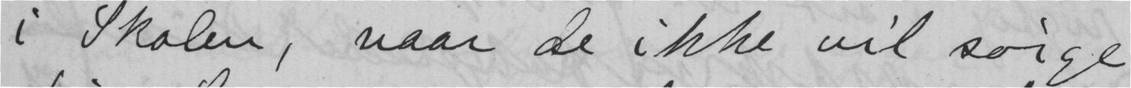i Skolen, naar de ikke vil sørge
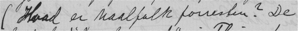(Hvad er maalfolk forresten? De
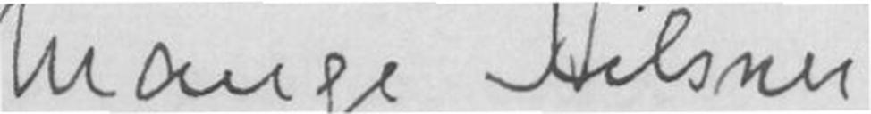Mange Hilsner
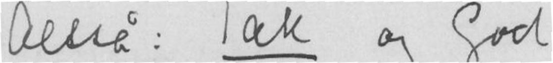Altså: Tak og God
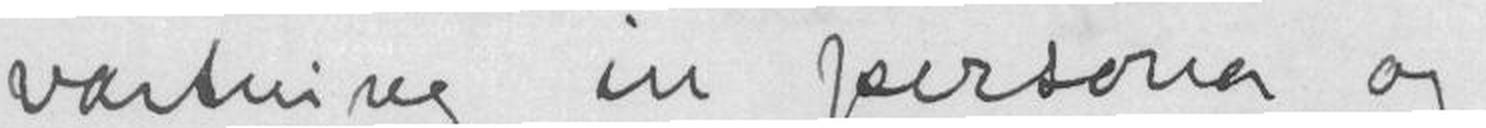vartning in persona og
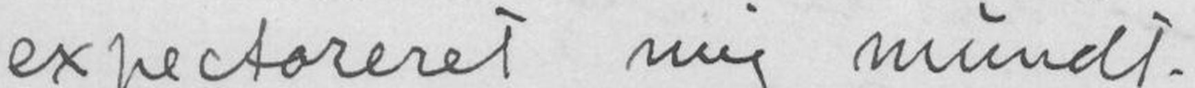expectoreret mig mundt-
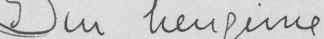Din hengivne
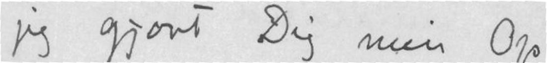jeg gjort Dig min Op
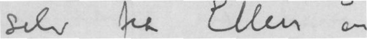selv fra Ellen og
